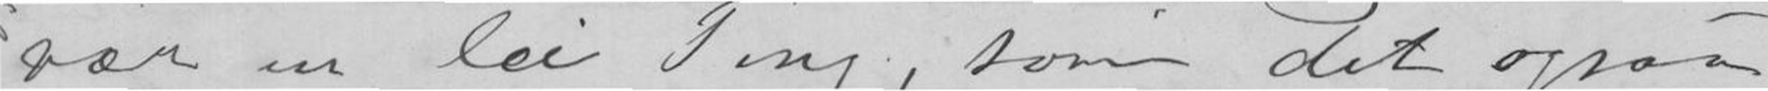være en lei Ting, som det ogsaa
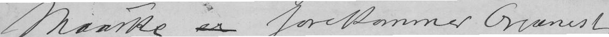Maaske forekommer Organist.
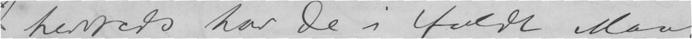hersteds har De i fulde Maal,
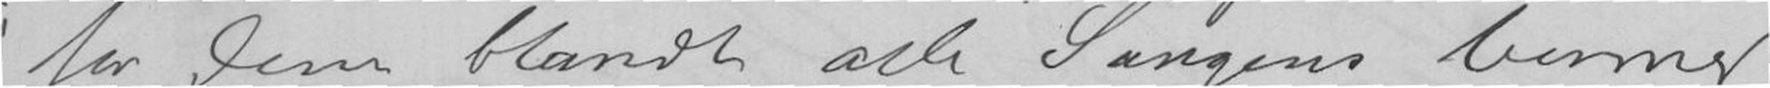for Dem blandt alle Sangens Venner
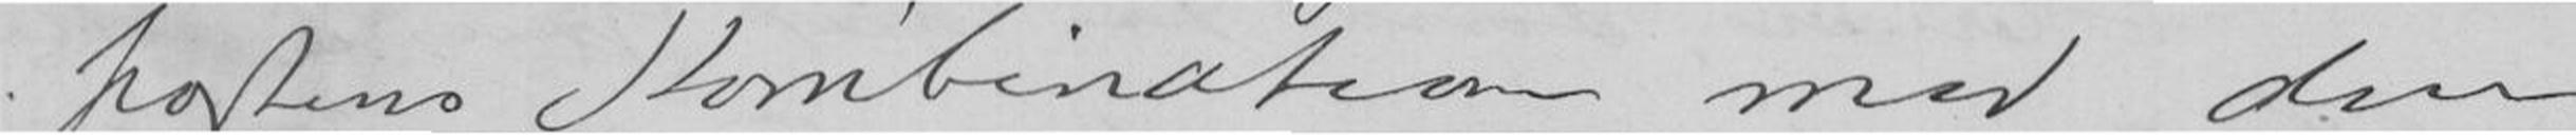postens Kombinationer med den
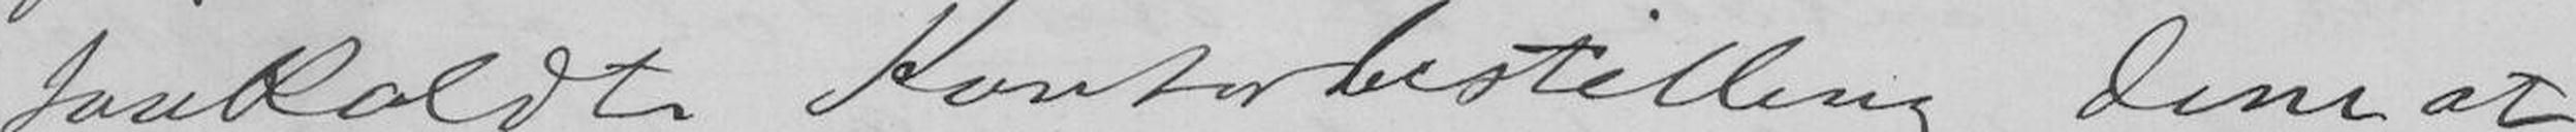saakaldte Kontorbestilling dem at
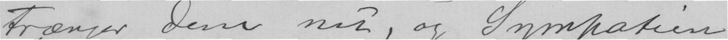Trænger Dem nu, og Sympatien
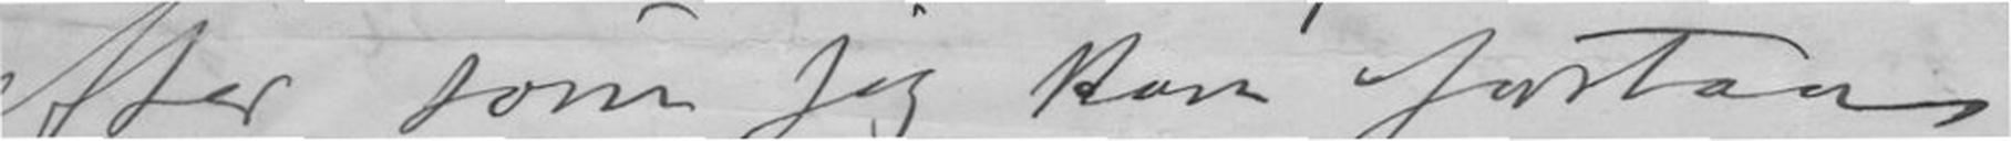efter som jeg kan forstaa.
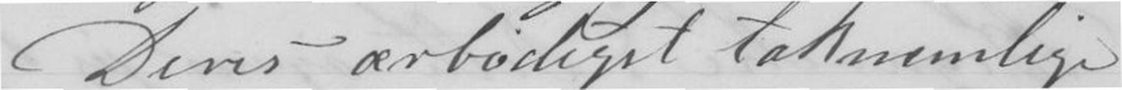Deres ærbødigst taknemlige
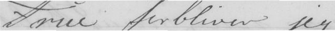Frue forbliver jeg
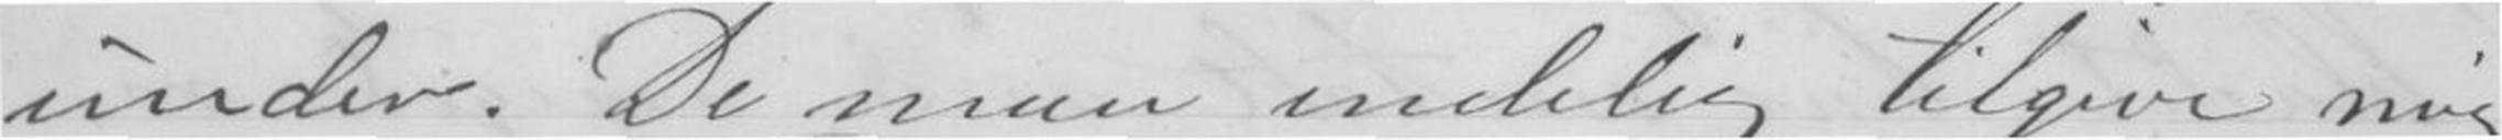under. De maa endelig tilgive mig
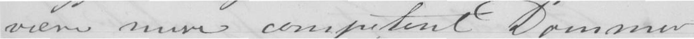være mere competent Dommer
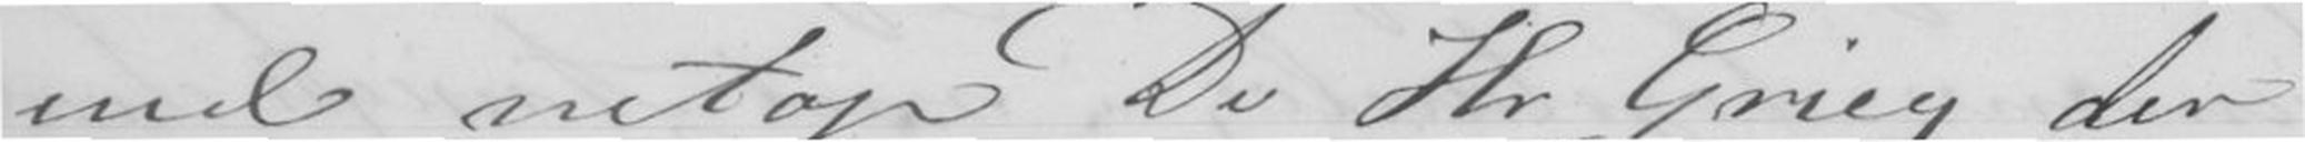end netop De Hr Grieg der
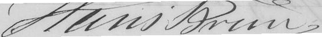Hans Brun
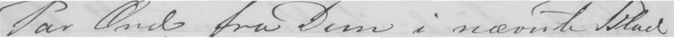Par Ord fra Dem i nævnte Blad
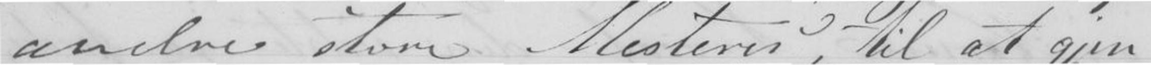andre store Mesters, til at gjen-
